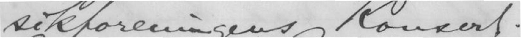sikforeningens Konsert.
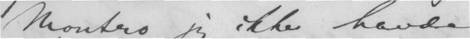Montro jeg ikke havde
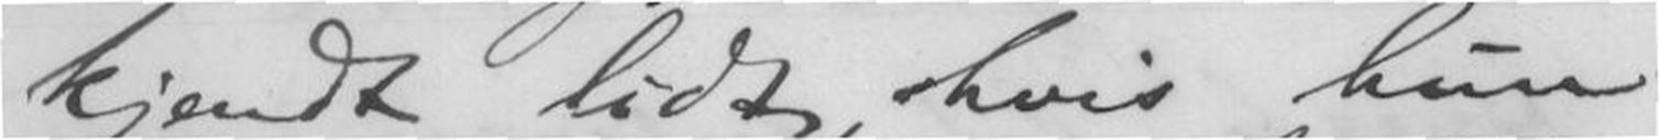kjendt lidt, hvis hun
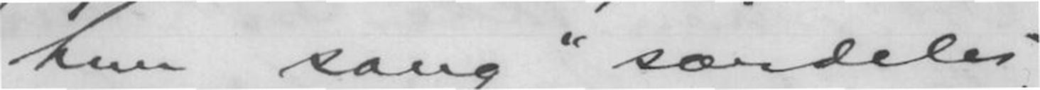hun sang "særdeles
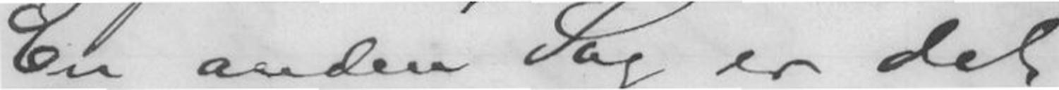En anden Sag er det,
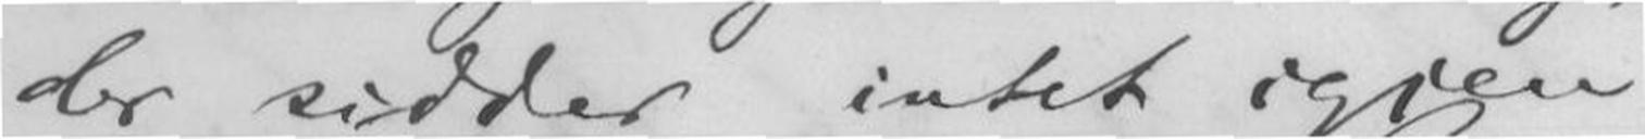der sidder intet igjen
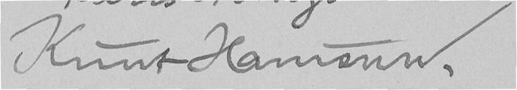Knut Hamsun.
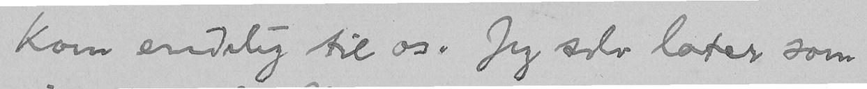kom endelig til os. Jeg selv later som
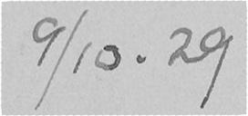9/10. 29
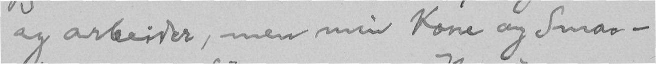og arbeider, men min Kone og Smaa-
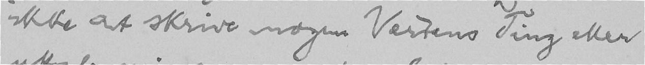ikke at skrive nogen Verdens Ting eller
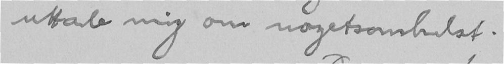uttale mig om nogetsomhelst.
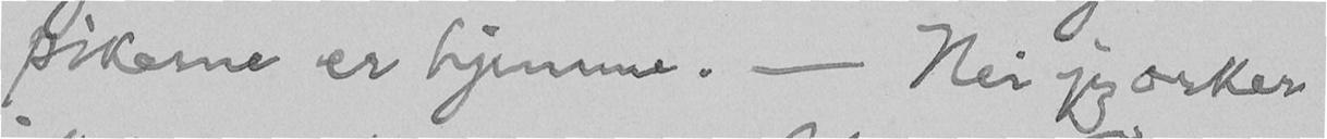pikerne er hjemme. - Nei jeg orker
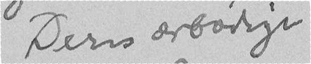Deres ærbødige
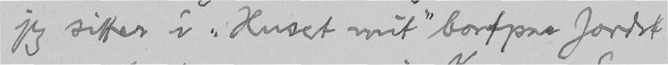jeg sitter i "Huset mit" bortpaa Jordet
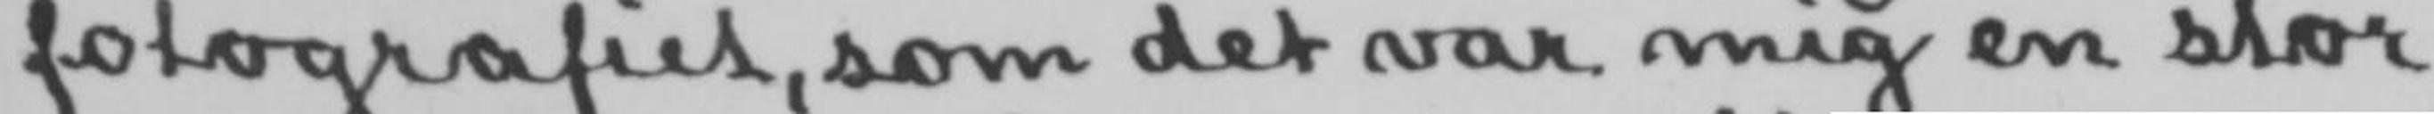fotografiet, som det var mig en stor
